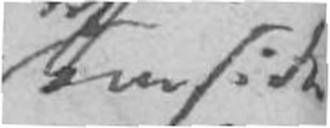omsider
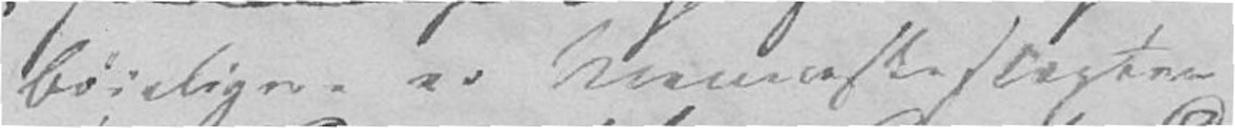bøieligere er Menneskeslægten
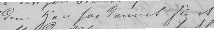den. Han har dannet sig et
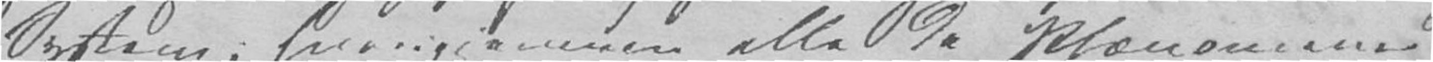System; hvorigjennem alle de Phænomener
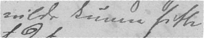vilde kunne hitte
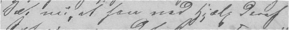Sæt nu, at han ved Hjelp deraf
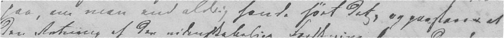paa, om man end aldrig havde hørt det, og paastaaer at
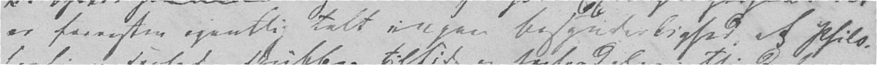er forresten egentlig talt ingen Besynderlighed, at Philo-
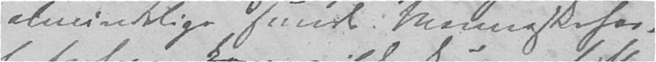almindelige sunde Menneskefor-
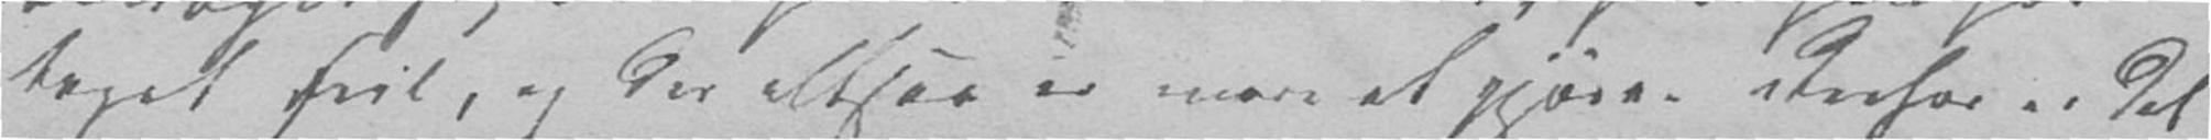taget feil, og der altsaa er mere at gjøre. Derfor er det
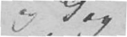og dog
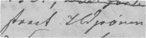staaet Ildprøven
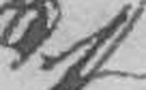der
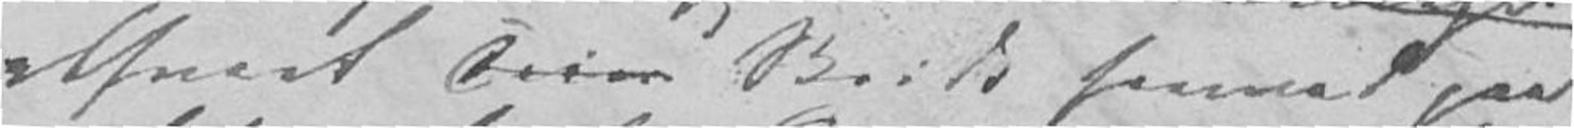ethvert Skridt fremad paa
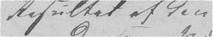Resultat af den
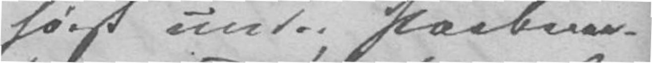først under Paaberaa-
